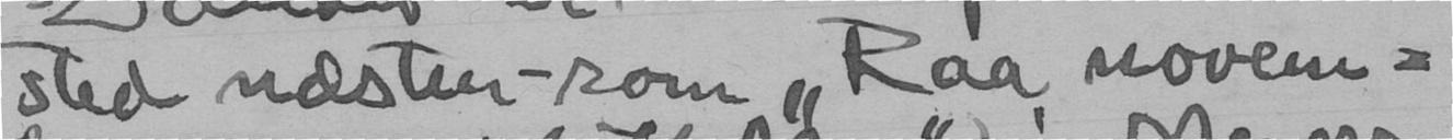sted næsten - som "Raa novem-
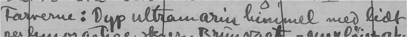Farverne: Dyp ultramarin himmnel med lidt
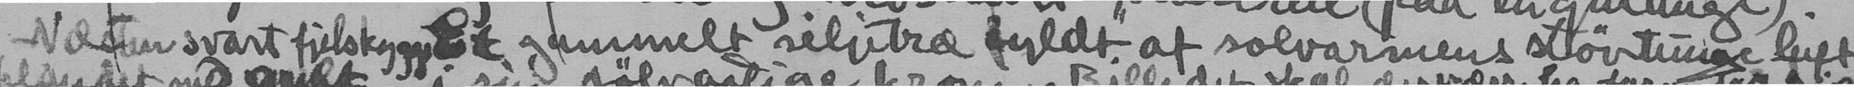Næsten svart fjelskygge. Et gammelt siljetræ fyldt af solvarmens støvtunge luft
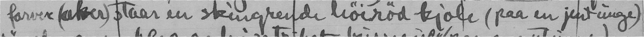farver (aker) staar en skingrende høirød kjole (paa en jentunge)
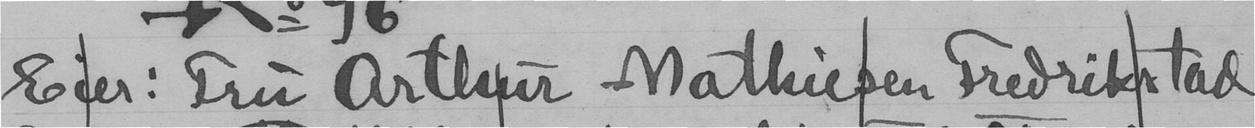Eier: Fru Arthur Mathiesen Fredrikstad
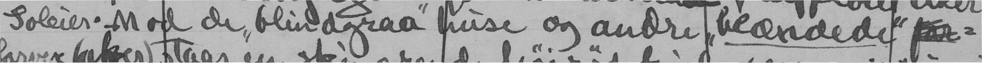Soleier Mod de "blindgraa" huse og andre "beændede"
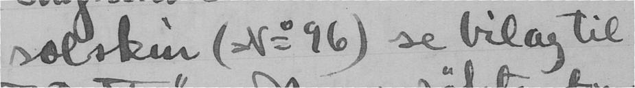solskin (No 96) se bilag til
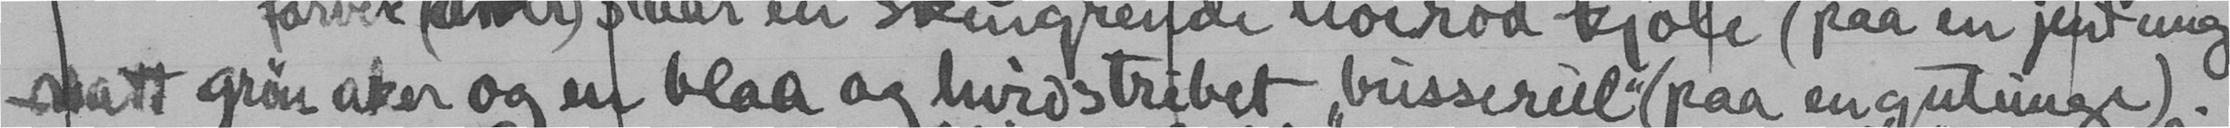Matt grøn aker og af blaa og hvidtribet "busserul"- (paa en gutunge).
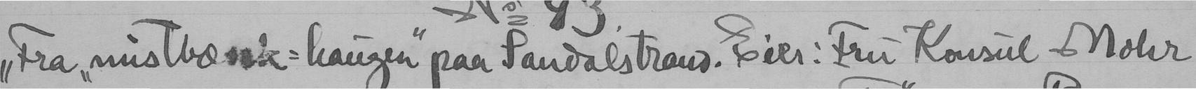"Fra "mistbænk-haugen" paa Sandalstrand. Eier: Fru Konsul Mohr
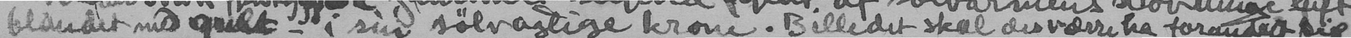blandet med gult - i sin sølvagtige krone. Billidet skal desværre ha forandret sig.
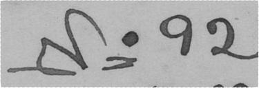No 92
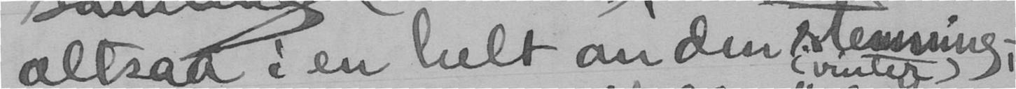altsaa i en helt anden stemning,-
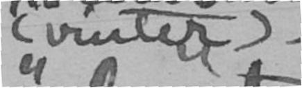(vinter)
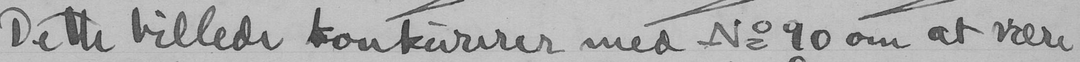Dette billede konkurerer med No 90 om at være
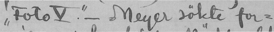"Foto V" - Meyer søkte for-
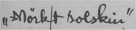"Mørkt solskin"
